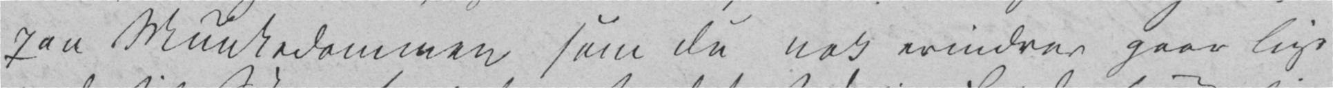paa Munkedammen som Du nok erindrer gaar lige ned til Søen
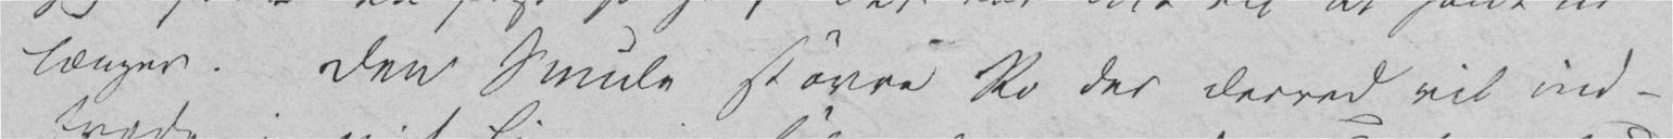længer. Den Smule større Ro der derved vil ind-
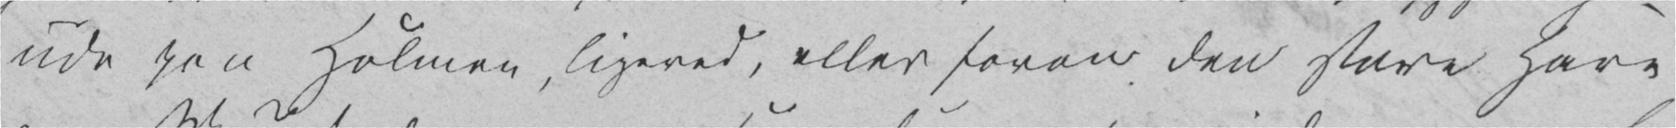ude paa Holmen, ligeved, eller foran den store Have
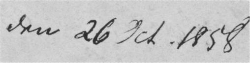Den 26 Oct. 1858
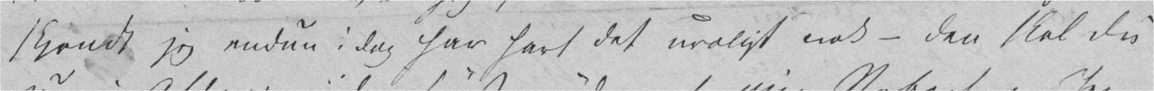skjønt jeg endnu i Dag har havt det uroligt nok – den skal Du
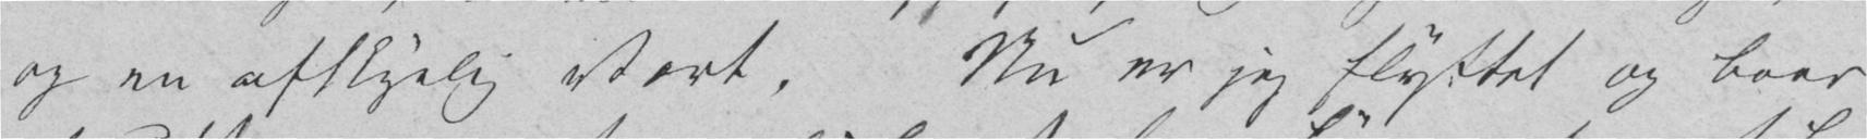og en afskyelig Vært. Nu er jeg flyttet og boer
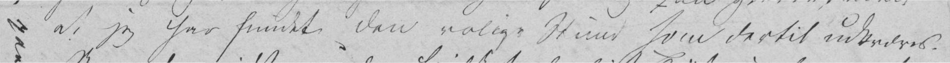at jeg har fundet den rolige Stund som dertil udkræves.
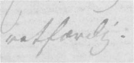retfærdig.
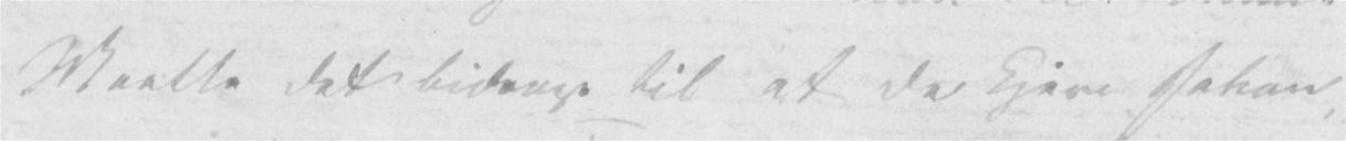Maatte det bidrage til at De kjere Johan,
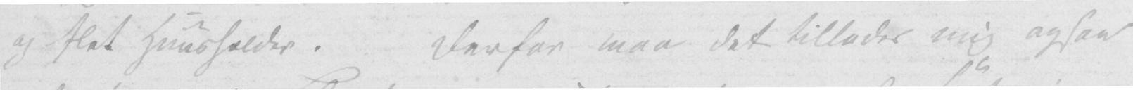og slet Huusholder. Derfor maa det tillades mig ogsaa
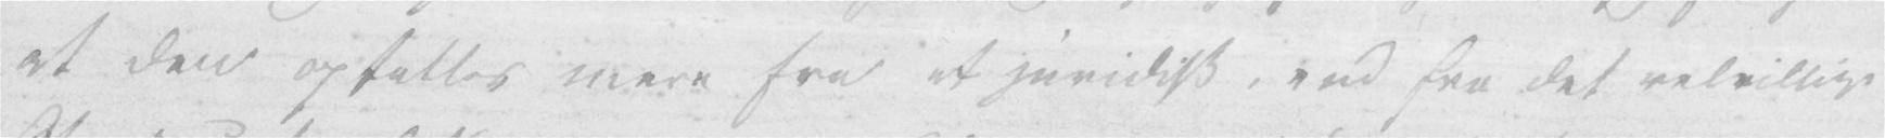at den omfattes mere fra et juridisk, end fra det velvillige
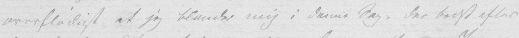overflødigt at jeg blander mig i denne Sag, der bedst efter
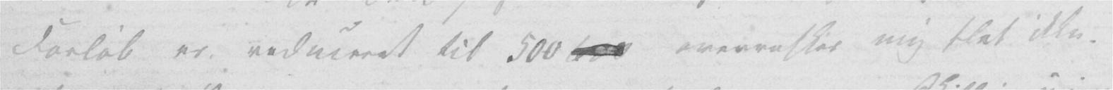Forløb er reduceret til 500 600 overrasker mig slet ikke.
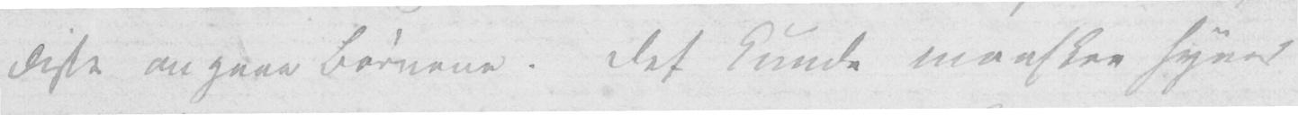disse angaae Børnene. Det kunde maaskee synes
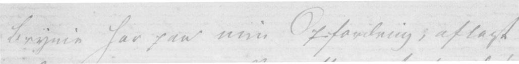Brynie har paa min Opfordring, aflagt
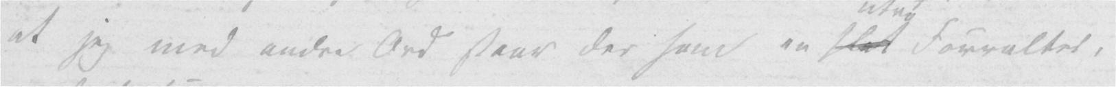at jeg med andre Ord staar der som en slet Forvalter,
